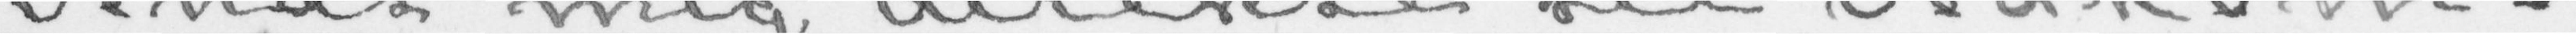vendt mig direkte til vedkom-
